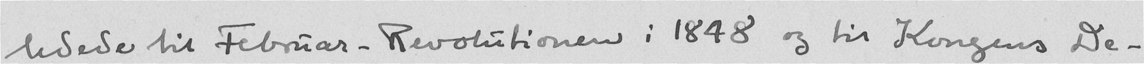ledede til Februar-Revolutionen i 1848 og til Kongens De-
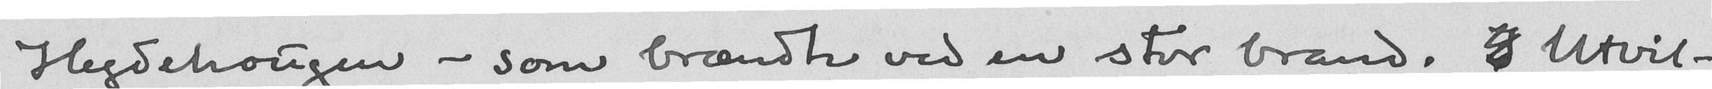Hegdehougen - som brændte ved en stor brand. I Utvil-
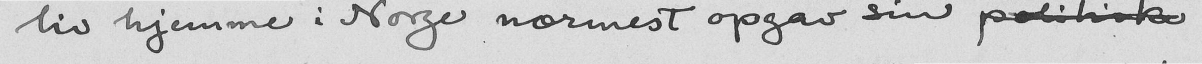liv hjemme i Norge nærmest opgav sin politiske
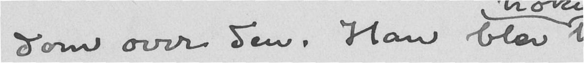dom over den. Han blev
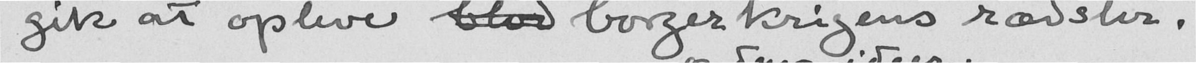gik at opleve blod borgerkrigens rædsler.
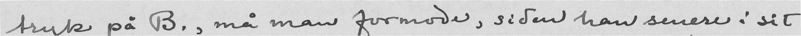tryk på B., må man formode, siden han senere i sit
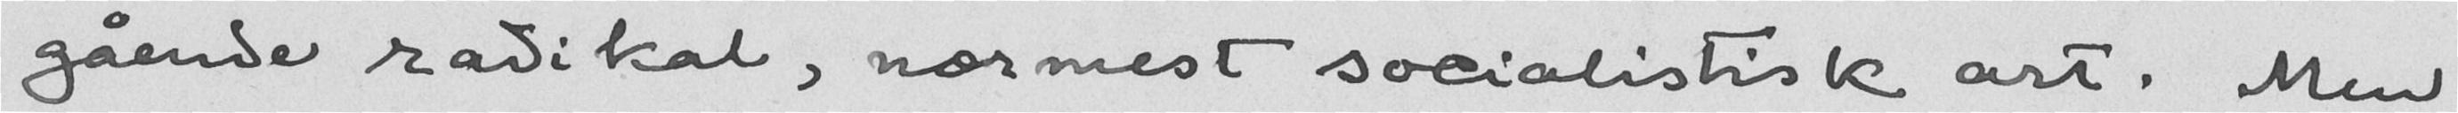gående radikal, nærmest socialistisk art. Men
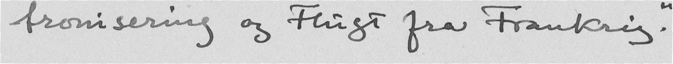tronisering og Flugt fra Frankrig."
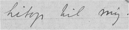hitop til mig.
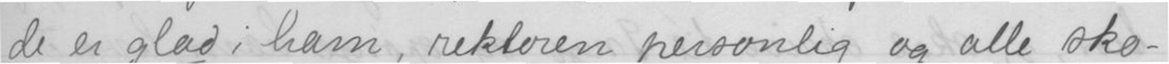de er glad i ham, rektoren personlig og alle sko-
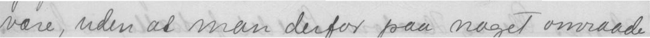være, uden at man derfor paa noget omraade
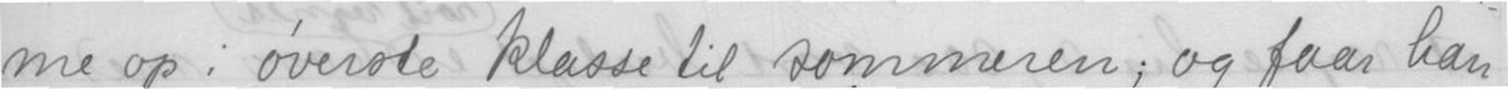me op i øverste klasse til sommeren; og faar han
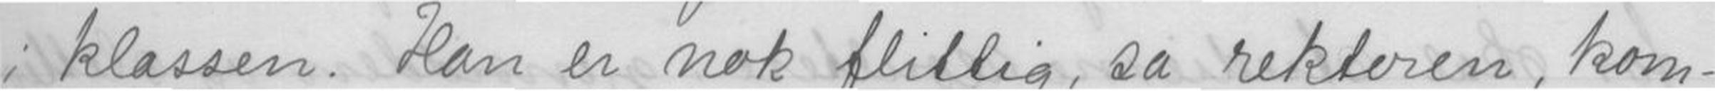i klassen. Han er nok flittig, sa rektoren, kom-
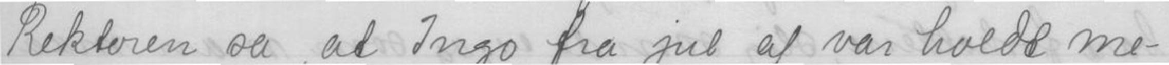Rektoren sa, at Ingo fra jul af var holdt me-
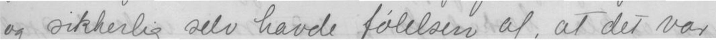og sikkerlig selv havde følelsen af, at det var
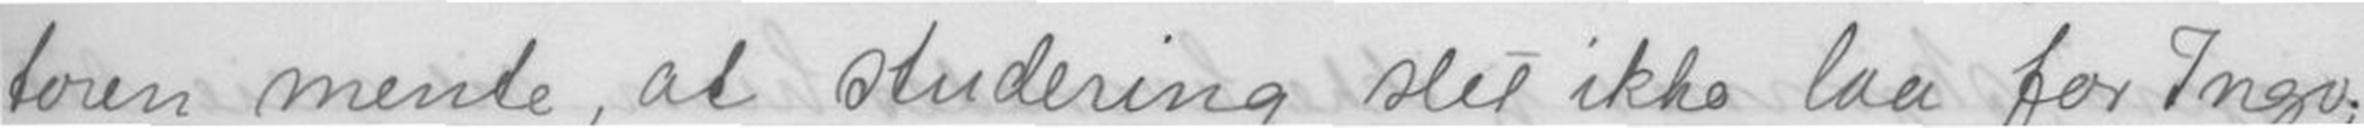toren mente, at studering slet ikke laa for Ingo;
